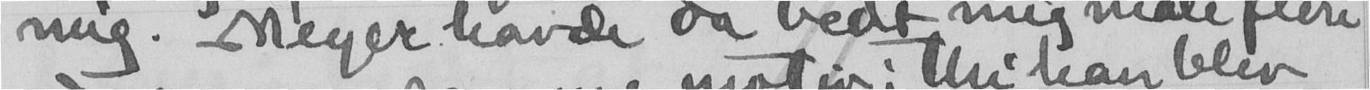mig. Meyer havde da bedt mig male flere
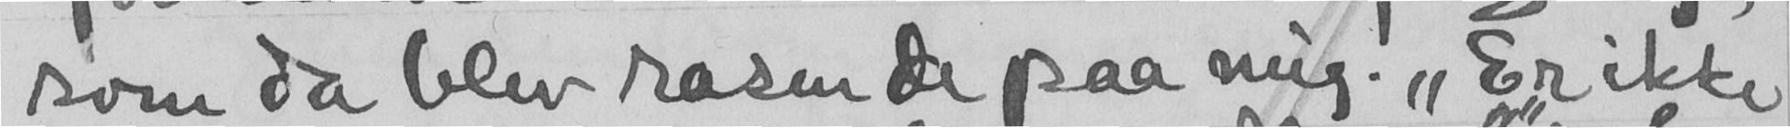som da blev rasende paa mig. "Er ikke
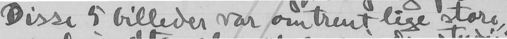Disse 5 billeder var omtrent lige store,
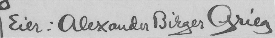Eier: Alexander Birger Grieg
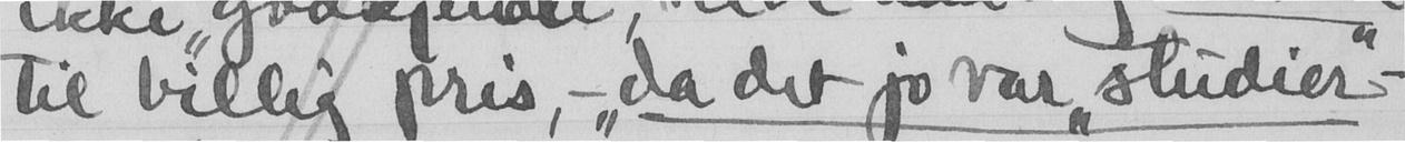til billig pris, - "da det jo var "studier -
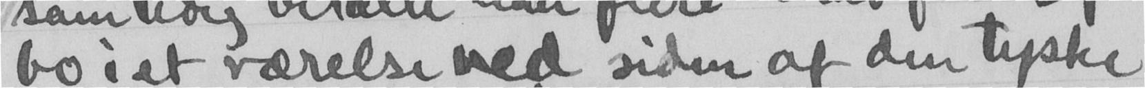bo i et værelse ved siden af den tyske
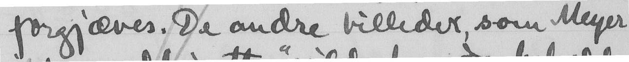forgjæves. De andre billeder som Meyer
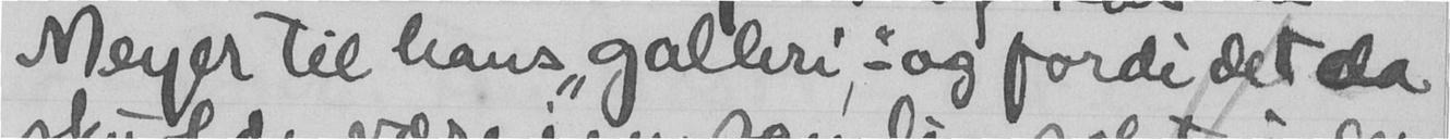Meyer til hans "galleri" - og fordi det da
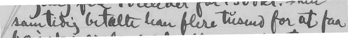samtidig betalte han flere tusend for at faa
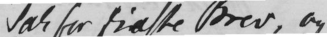Tak for sidste Brev, og
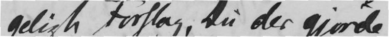geligt Forslag, Du der gjorde
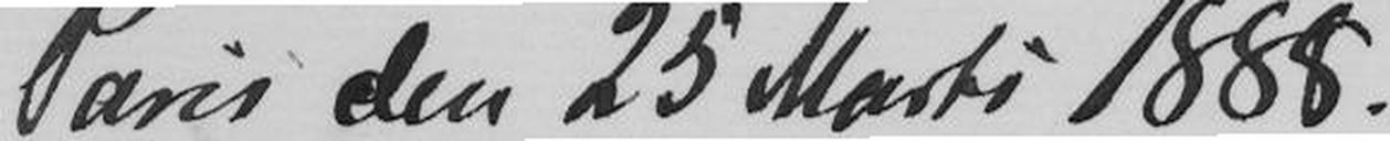Paris den 25 Marts 1888.
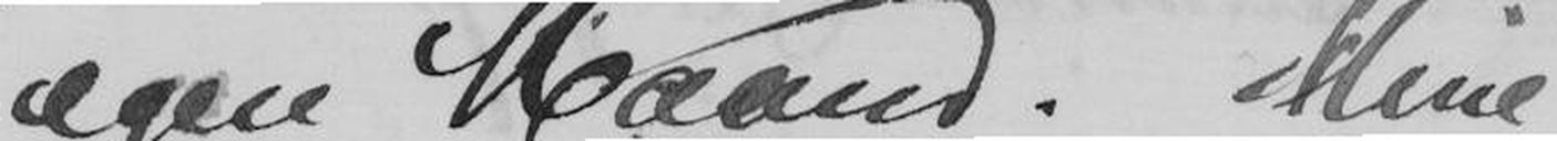egen Haand. Mine
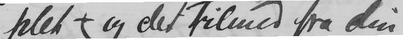plet og det tilmed fra din
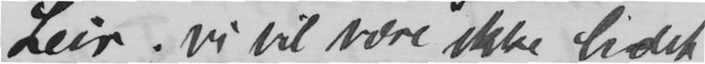Leir; vi vil være ikke lidet
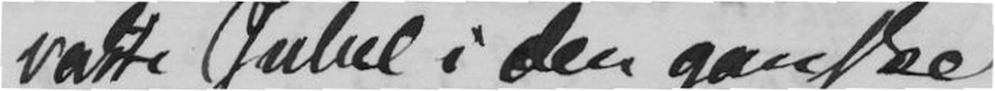rætte Jubel i den ganske
